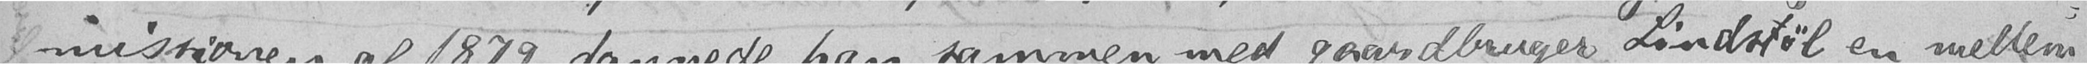missionen af 1879 dannede han sammen med gaardbruger Lindstøl en mellem-
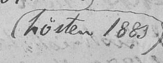høsten 1883
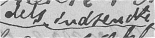dels indsendte
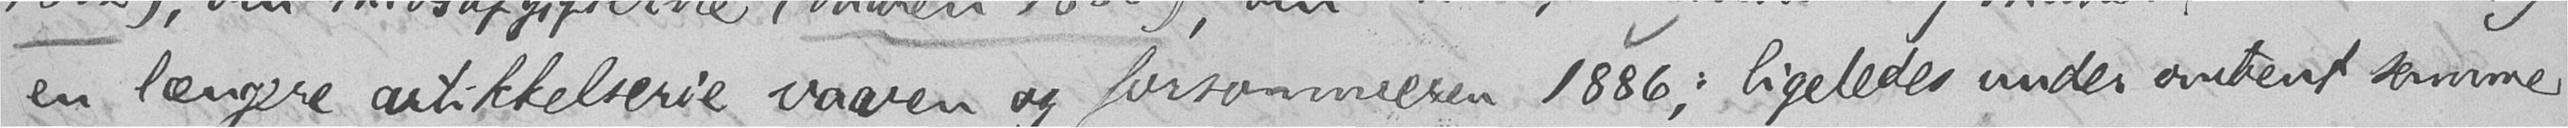en længere artikkelserie vaaren og forsommeren 1886; ligeledes under omtrent samme
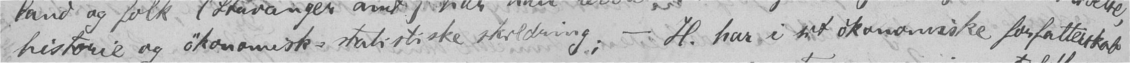historie og økonomisk-statistiske skildring; - H. har i sit økonomiske forfatterskab
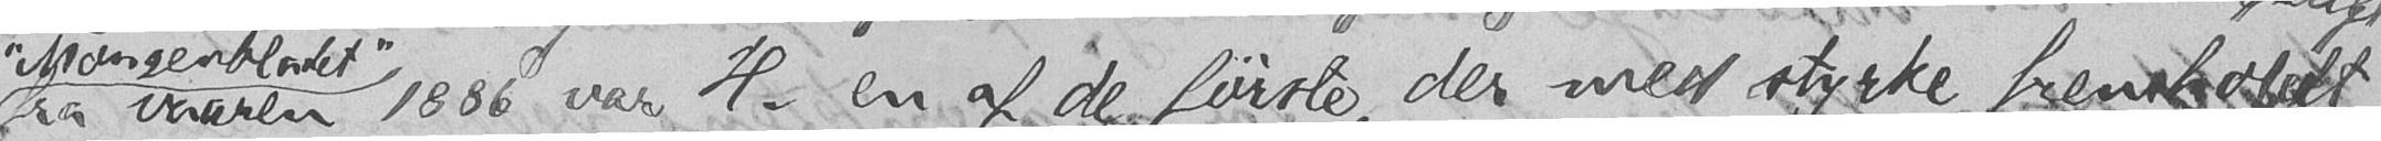fra vaaren 1886 var H. en af de første, der med styrke fremholdt
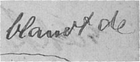blandt de
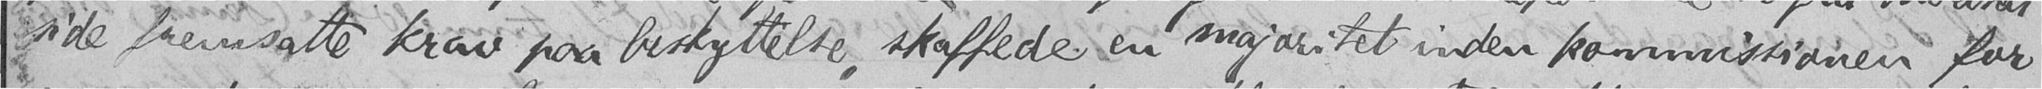side fremsatte krav paa beskyttelse, skaffede en majoritet inden kommissionen for
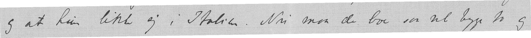og at hun likte sig i Italien. Nu maa de leve saa vel begge to og
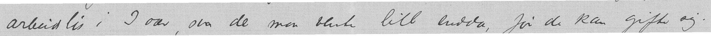arbeidsløs i 3 aar, saa de maa vente litt endda, før de kan gifte sig.
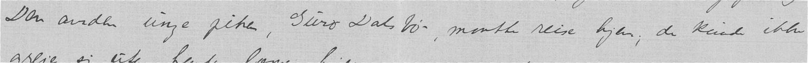Den anden unge piken, Guro Dalsbø, maatte reise hjem; de kunde ikke
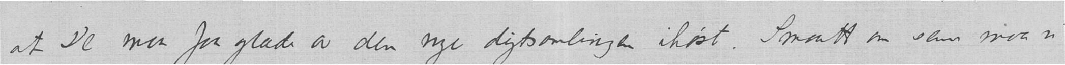at De maa faa glæde av den nye digtsamlingen ihøst. Smaatt om senn maa vi
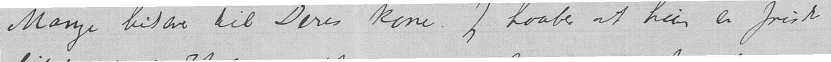Mange hilsener til Deres kone. Jeg haaber at hun er frisk
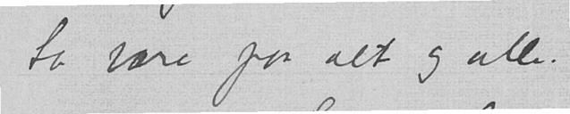ta vare paa alt og alle.
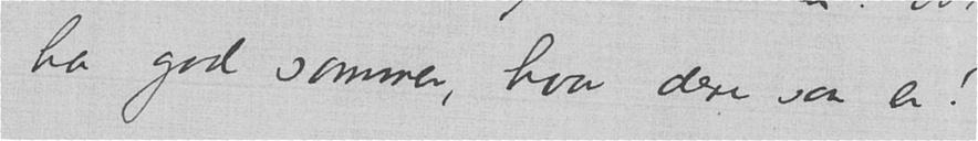ha god sommer, hvor dere saa er!
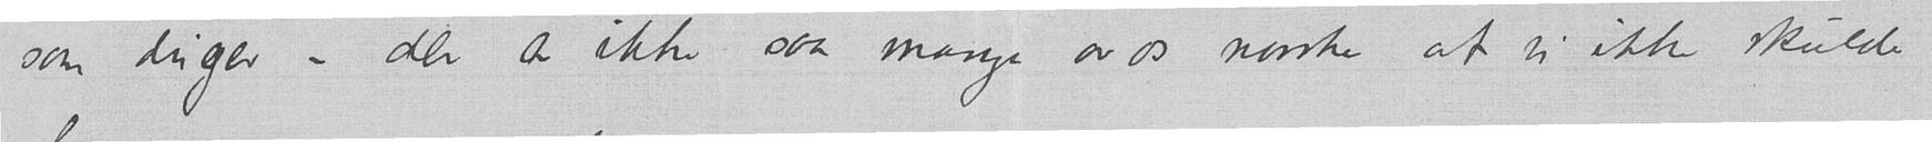som duger – der er ikke saa mange av os norske at vi ikke skulde
